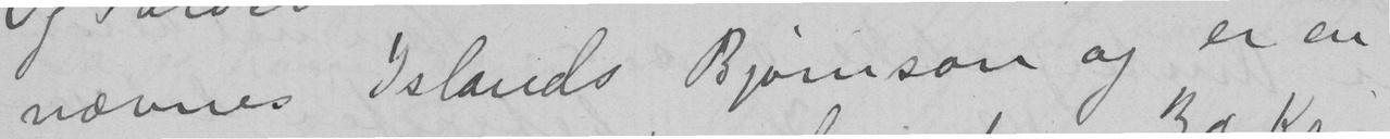nærnes Islands Bjørnson og er end
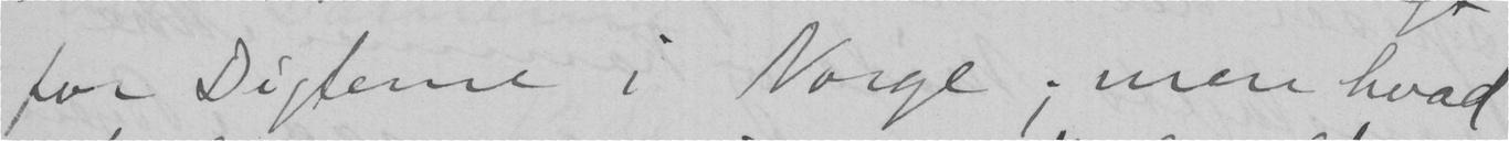for Digterne i Norge; men hvad
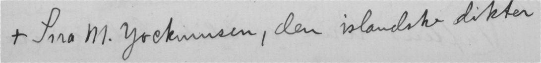x Sira M. Jockumsen, den islandske dikter
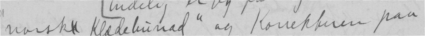"norske Klædebunad" og Korrekturen paa
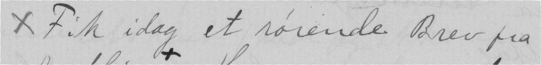x Fik idag et rørende Brev fra
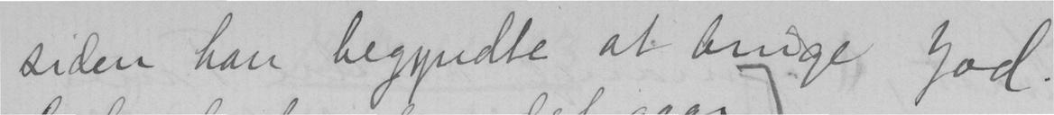siden han begyndte at bruge jod.
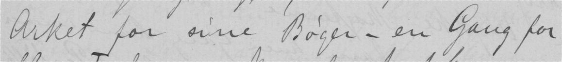arket for sine Bøger - en Gang for
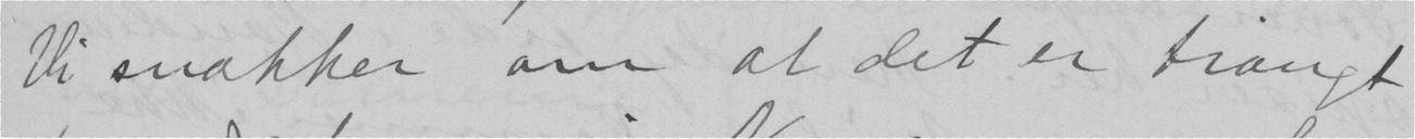Vi snakker om at det er trangt
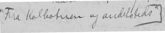"Fra Kolbotnen og andetsteds".
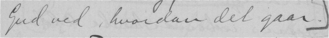Gud ved, hvordan det gaar.
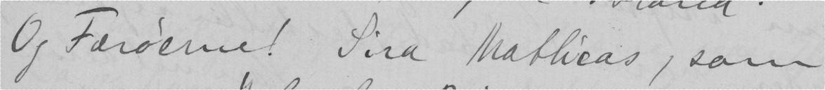Og Færøerne! Sira Mathias, som
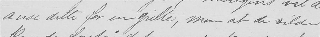anse dette for en grille, men at de vilde
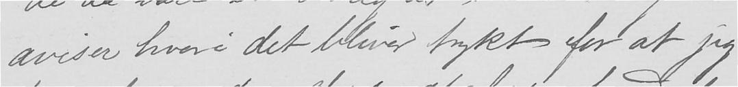aviser hvori det bliver trykt for at jeg
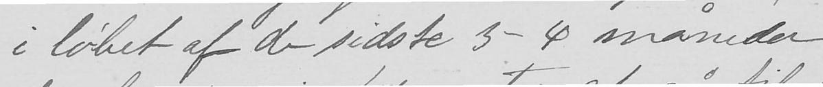i løbet af de sidste 3- 4 måneder
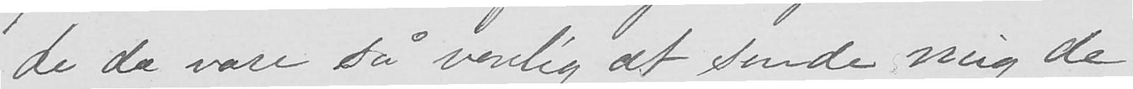de da være så venlig at sende mig de
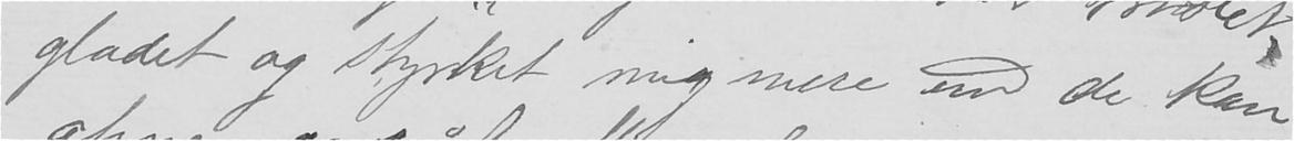glædet og styrket mig mere end de kan
